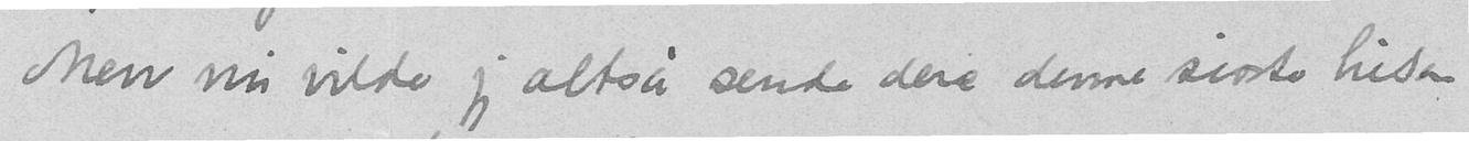Men nu vilde jeg altså sende dere denne sisste hilsen
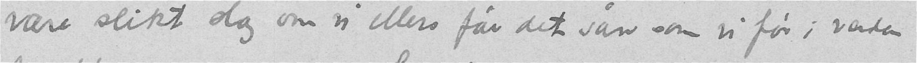være slikt slag om vi ellers får det sån som vi før i verden
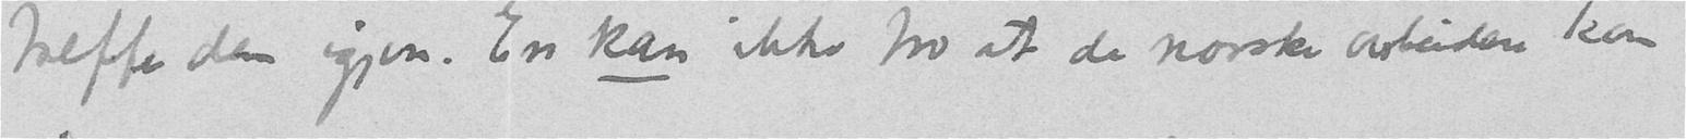treffe dem igjen. En kan ikke tro at de norske arbeidere kan
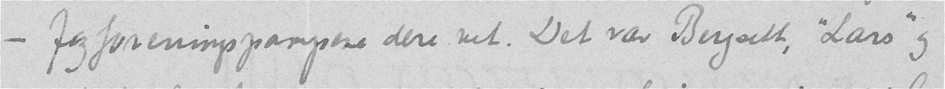- fagforeningspampene dere vet. Det var Bergsett, "Lars" og
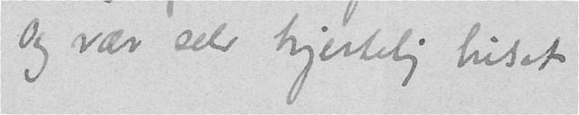Og vær selv hjertelig hilset
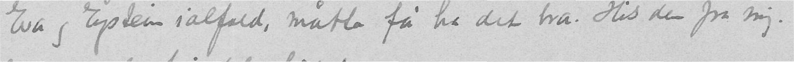Eva og Eystein ialfald, måtte få ha det bra. Hils dem fra mig.
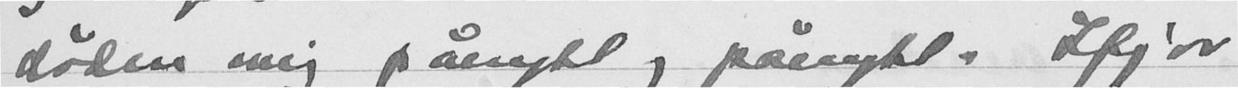døden mig pånytt og pånytt. Ifjor
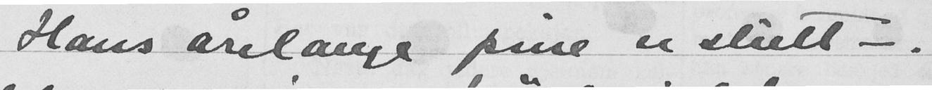Hans årelange pine er slutt -.
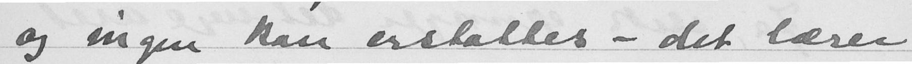og ingen kan erstattes - det lærer
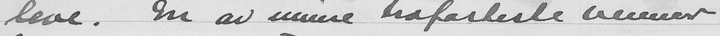leve. En av mine trofasteste venner
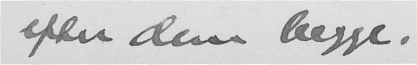efter dem begge.
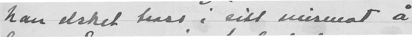han elsket tross i sitt mismot å
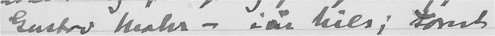Gustov Mahs - iår Nils; Først
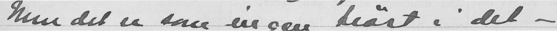Men det er som ingen trøst i det -
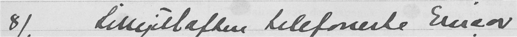8/6  Lillejulaften telefonerte Einar
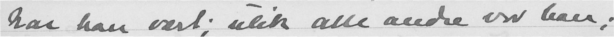har han vært; ulik alle andre var han;
